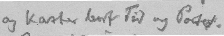og kaster bort Tid og Porto.
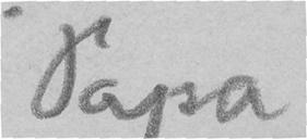Papa
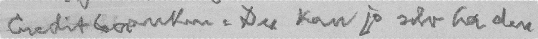Creditbanken. Du kan jo selv ha den
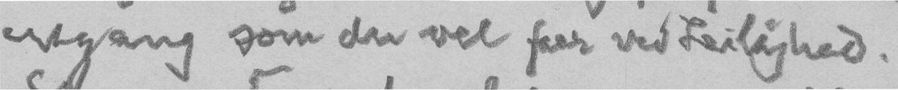engang som du vel faar ved Leilighed.
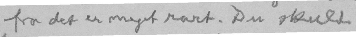fra det er meget rart. Du skulde
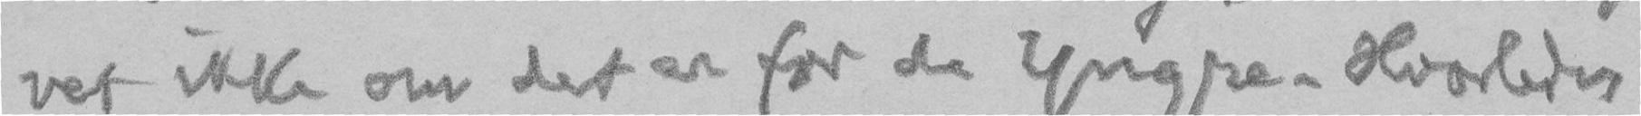vet ikke om det er for de yngre. Hvorledes
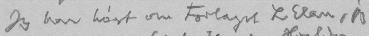Jeg har hørt om Forlaget L Elan, jeg
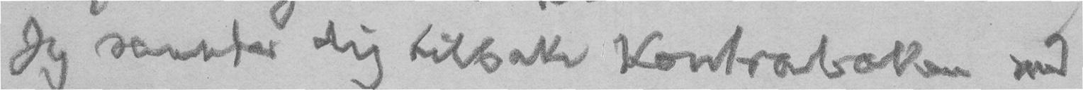Jeg sender dig tilbake Kontraboken med
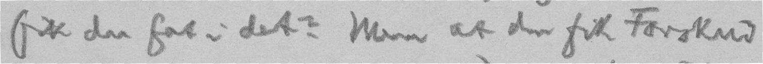fik du fat i det? Men at du fik Forskud
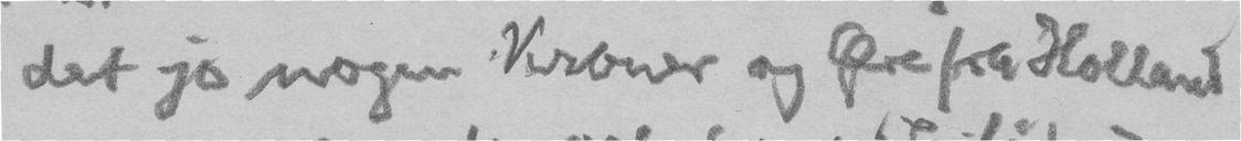det jo nogen Kroner og Øre fra Holland
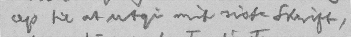op til at utgi mit siste Skrift,
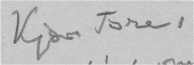Kjære Tore,
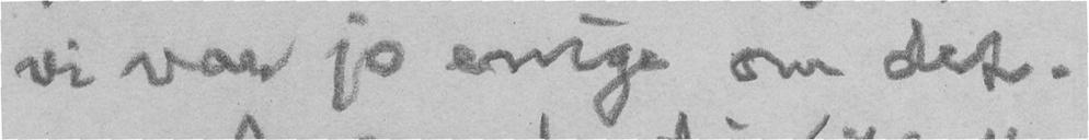vi var jo enige om det.
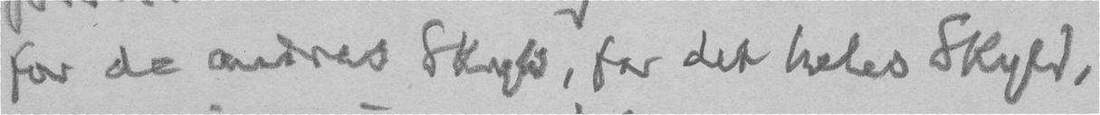for de andres Skyld, for det heles Skyld,
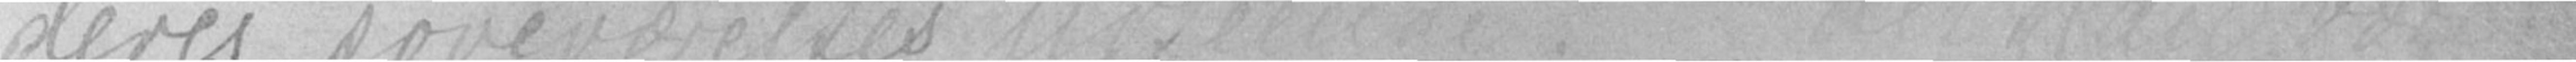deres soveværelses utseende;
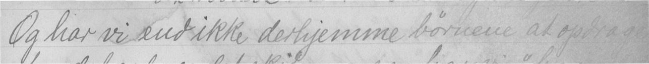Og har vi ænd ikke derhjemme børnene at opdrage
